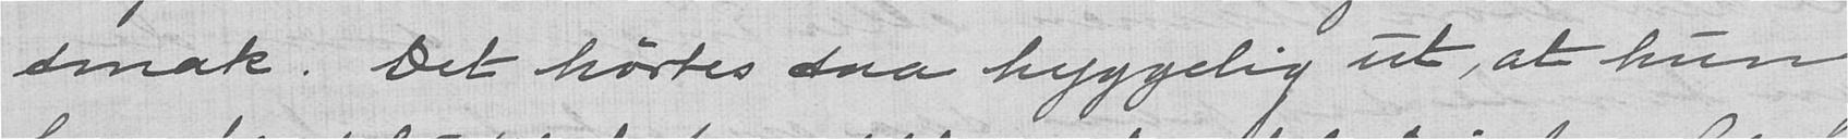smak. Det hørtes saa hyggelig ut, at hun
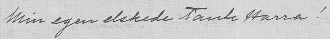Min egen elskede Tante Harra!
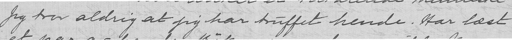Jeg tror aldrig at jeg har truffet hende. Har læst
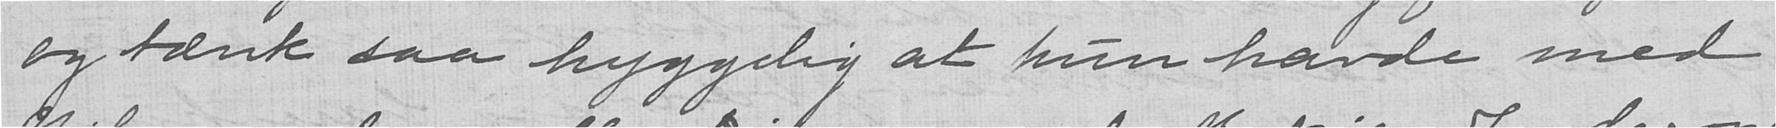og tænk saa hyggelig at hun havde med
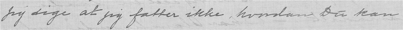jeg sige at jeg fatter ikke, hvordan Du kan
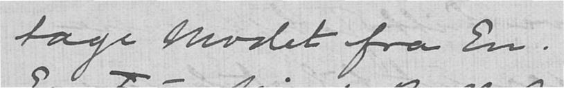tage modet fra En.
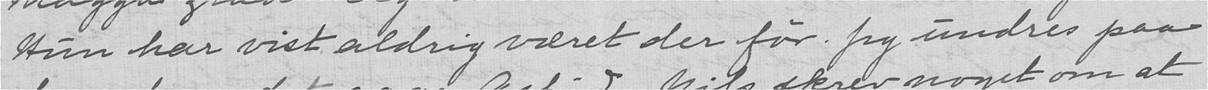Hun har vist aldrig været der før. Jeg undres paa
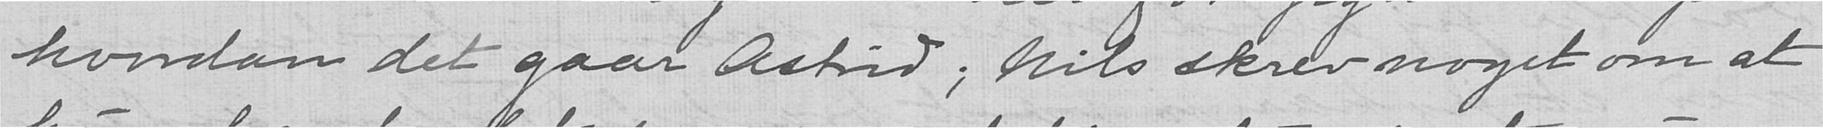hvordan det gaar Astrid; Nils skrev noget om at
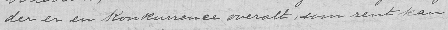der er en Konkurrence overalt, som rent kan
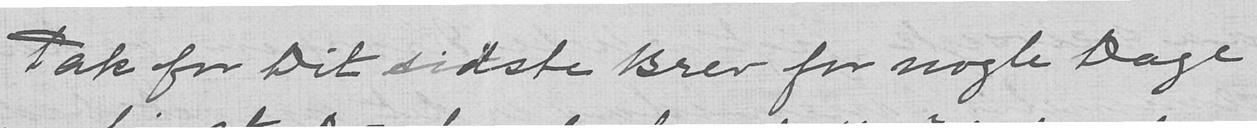Tak for Dit sidste Brev for nogle Dage
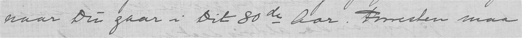naar Du gaar i Dit 80de Aar. Forresten maa
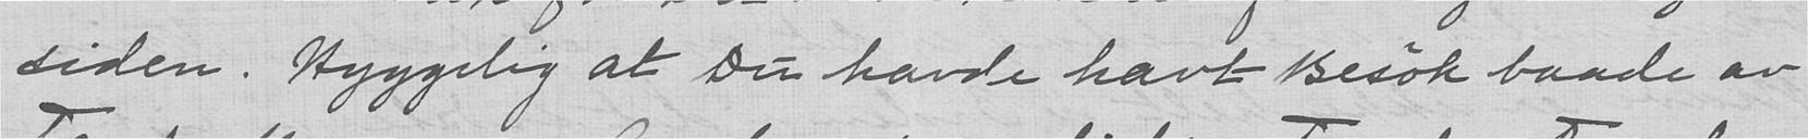siden. Hyggelig at Du havde havt besøk baade av
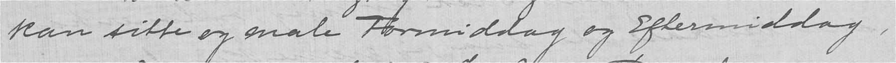kan sitte og male Formiddag og Eftermiddag,
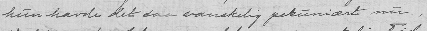hun havde det saa vanskelig pekuniært nu,
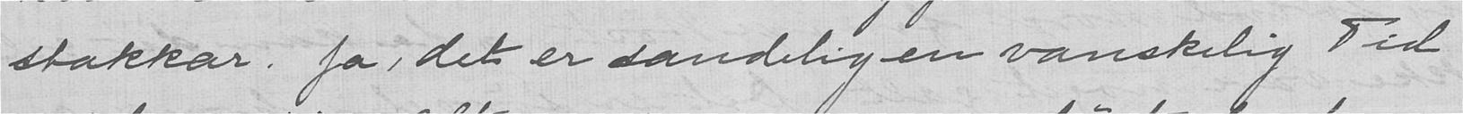stakkar. Ja, det er sandelig en vanskelig Tid
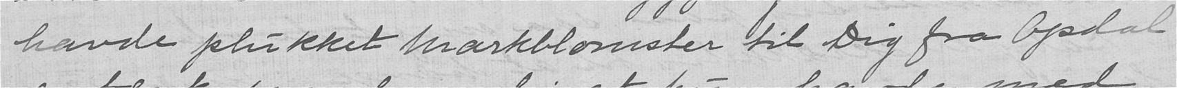havde plukket Markblomster til Dig fra Opdal,
